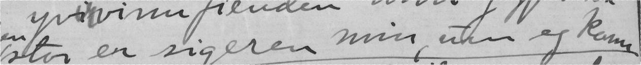stor er sigeren min, um eg kann
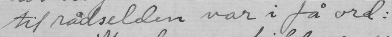til rådselden var i få ord:
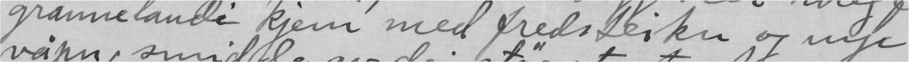grannelandi kjem med fredsteikn og nye
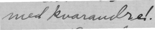med kvarandre!
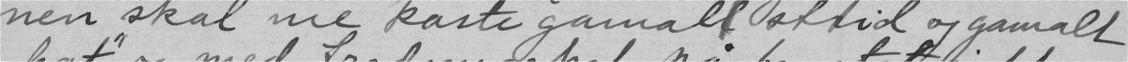nen skal me kaste gamalt strid og gamalt
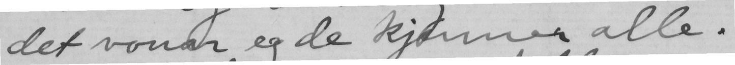det vonar eg de kjenner alle.
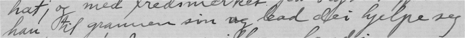han til grannen sin og bad dei hjelpe seg
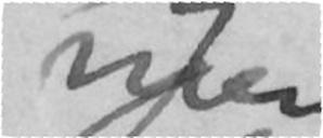men
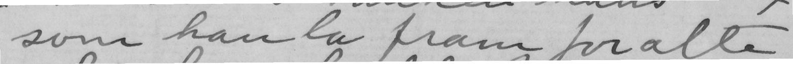som han la fram for alle
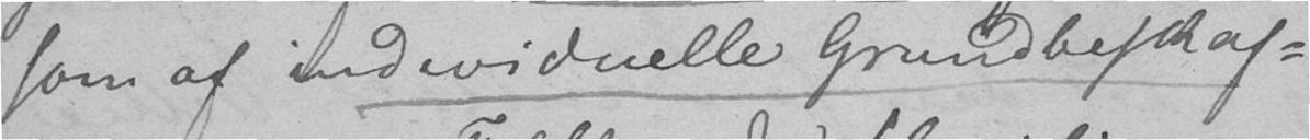som af individuelle Grundbeskaf-
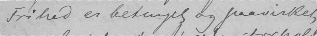Frihed er betinget og paavirket
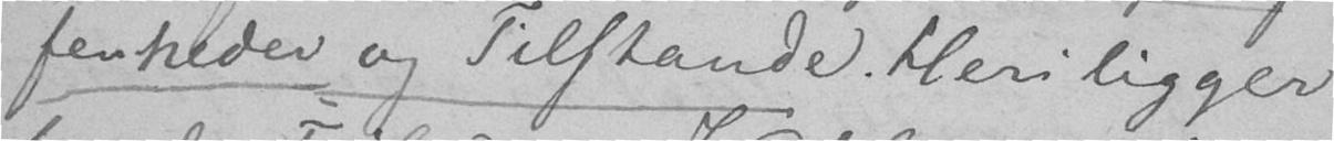fenheder og Tilstande. Heri ligger
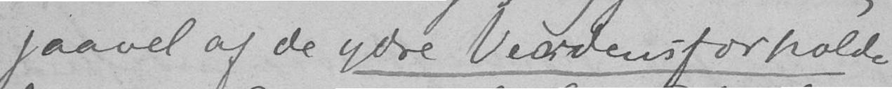saavel af de ydre Verdensforholde
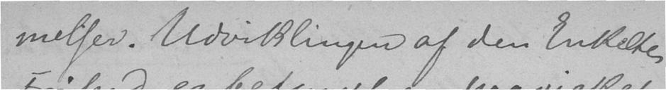melser. Udviklingen af den Enkeltes
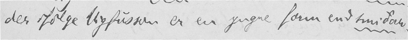der ifølge Vigfusson er en yngre form end smiðar.
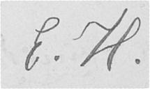E. H.
